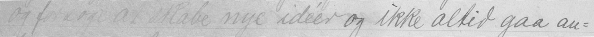og forsøge at skabe nye idéer og ikke altid gaa an-
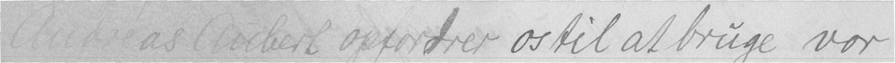Andreas Aubert opfordrer os til at bruge vor
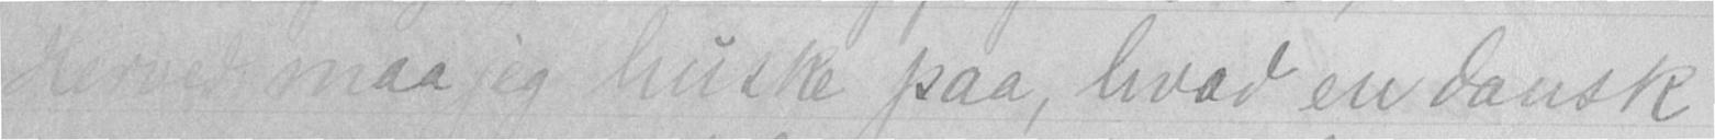Herved maa jeg huske paa, hvad en dansk
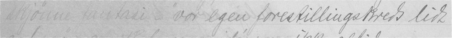skjønne fantasi - "vor egen forestillingskreds lidt
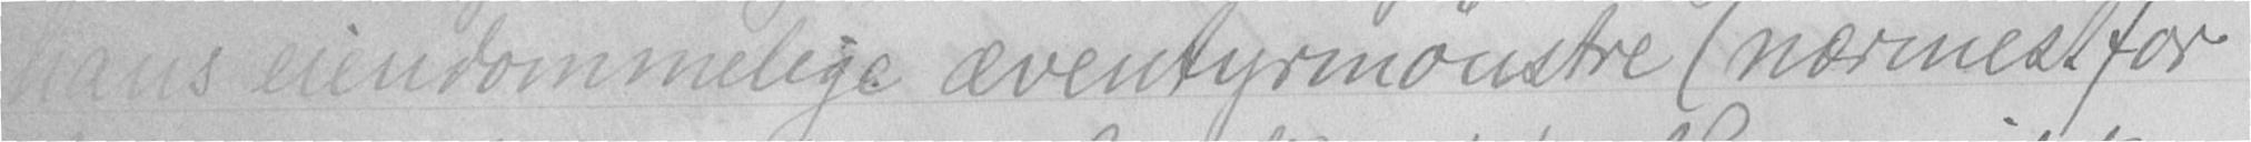hans eiendommelige æventyrmønstre (nærmest for
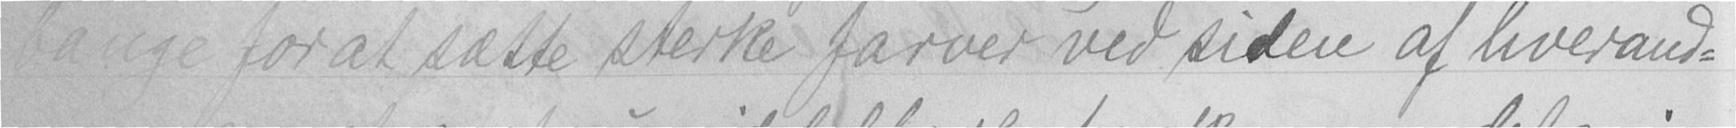bange for at sætte sterke farver ved siden af hverand-
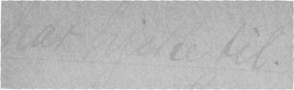har hjerte til.
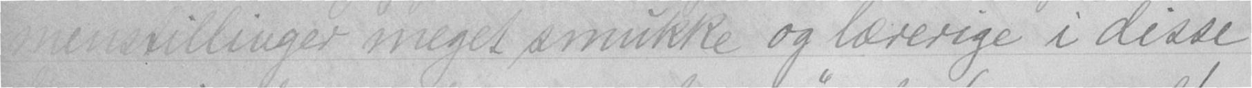menstillinger meget smukke og lærerige i disse
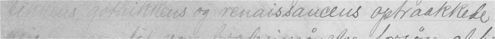tikkens, gothikkens og renaissancens optraakkede
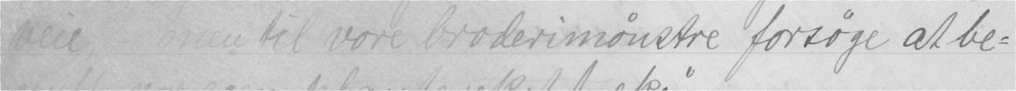veie, - men til vore broderimønstre forsøge at be-
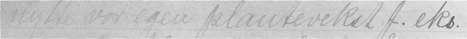nytte vor egen plantevekst f. eks."
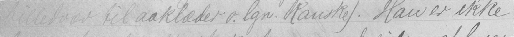billedvæv til aaklæder o. lgn. kanske). Han er ikke
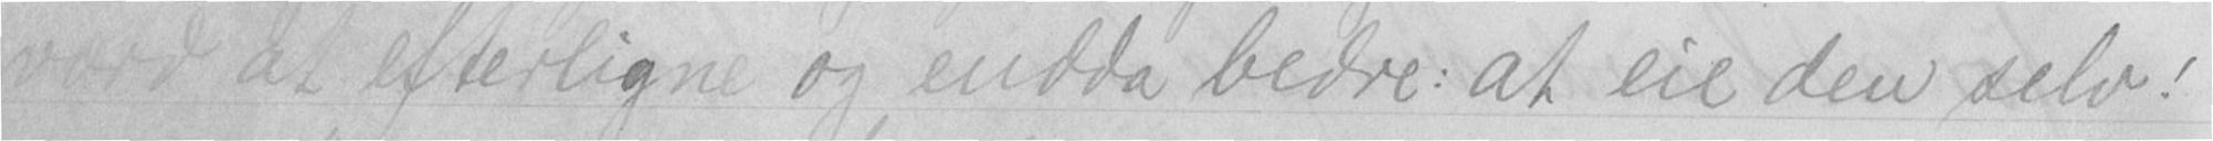værd at efterligne og endda bedre: at eie den selv!
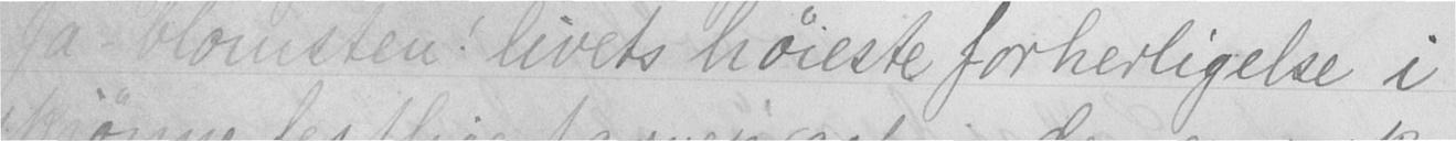Ja - blomsten! livets høieste forherligelse i
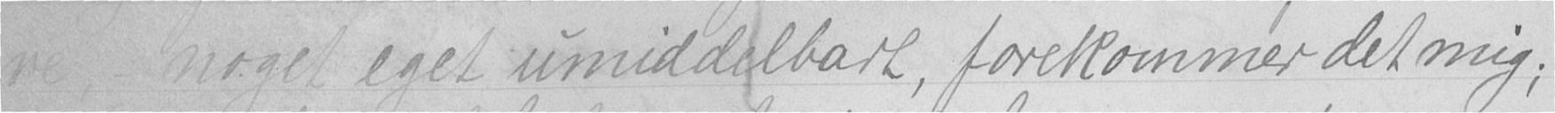re, - noget eget umiddelbart, forekommer det mig;
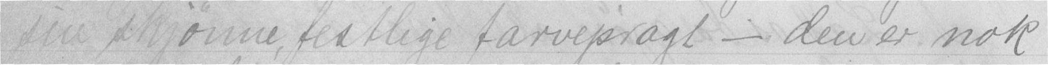sin skjønne, festlige farvepragt - den er nok
